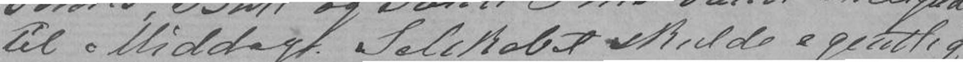til Middag. Selskabet skulde egentlig
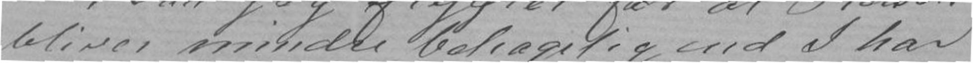bliver mindre behagelig end I har
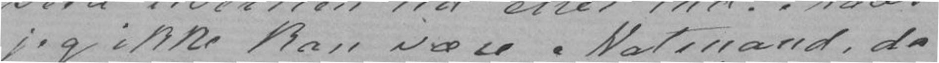jeg ikke kan være Natmand, da
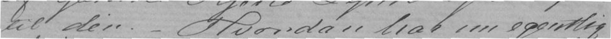til den. - Hvordan har nu egentlig
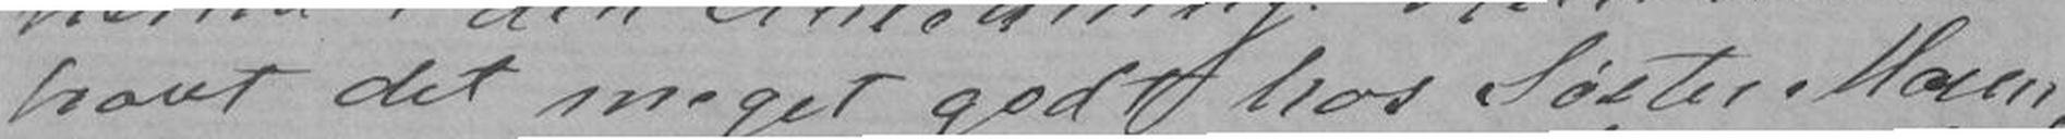havt det meget godt hos Søster Maren.
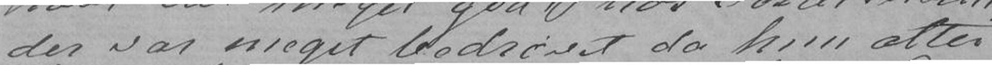der var meget bedrøvet da hun atter
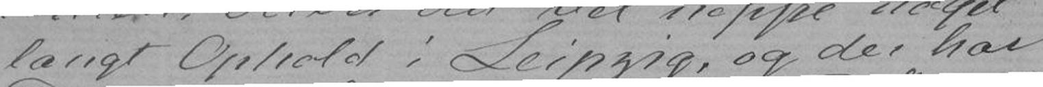langt Ophold i Leipzig, og der har
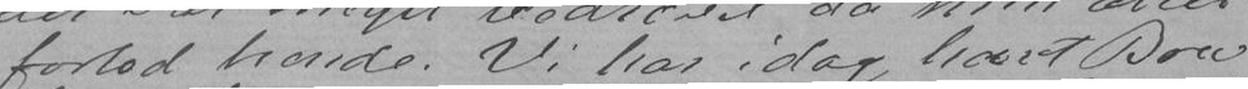forlod hende. Vi har idag havet Brev
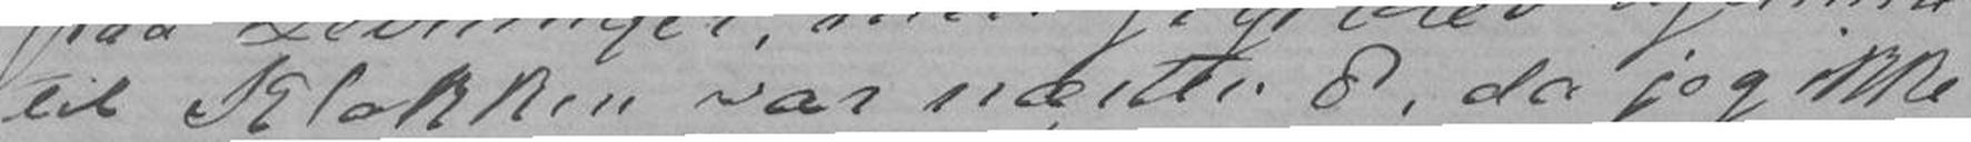til Klokken var næsten 8, da jeg ikke
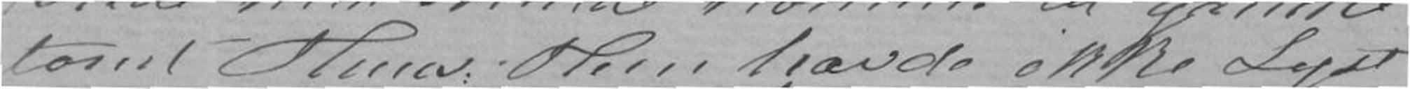tomt Huus. Hun havde ikke lyst
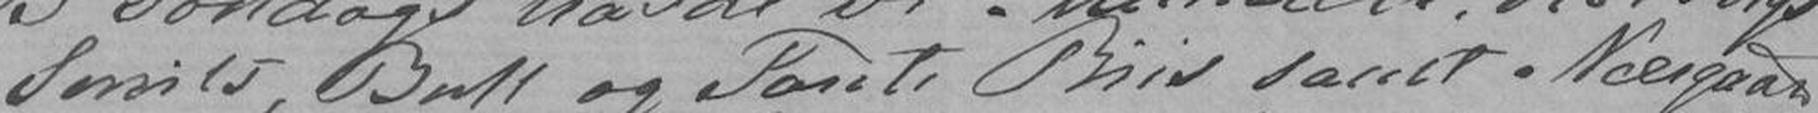Smits, Bull og Tante Riis samt Neergaard
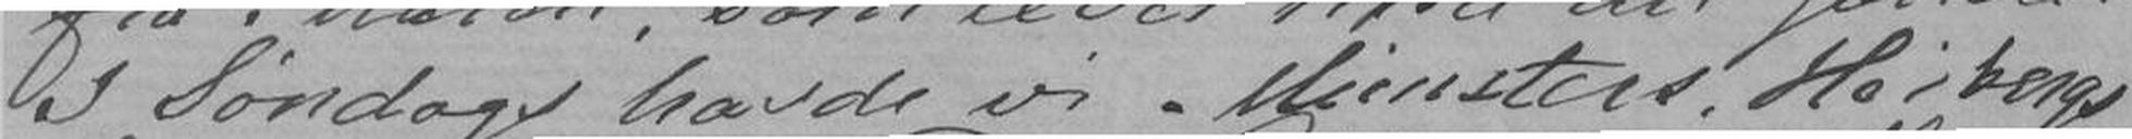I Søndags havde vi Münsters Heibergs
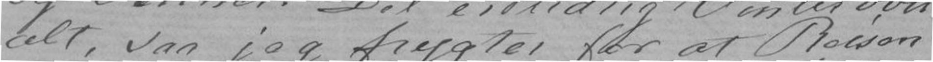alt, saa jeg frygter for at Reisen
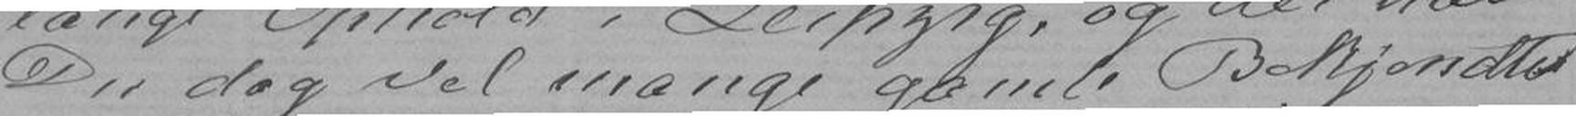Du dog vel mange gamle Bekjendte
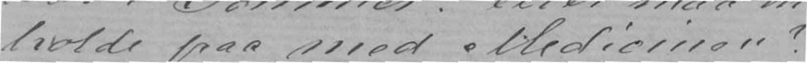holde paa med Mediciner?
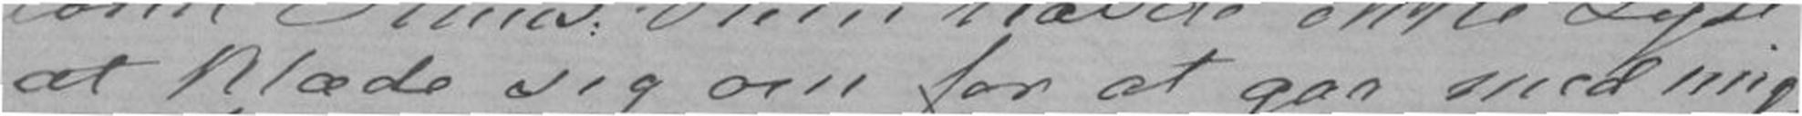at klæde sig om for at gaa med mig,
